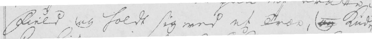Field og holdt sig ved et Træe,  Kud-
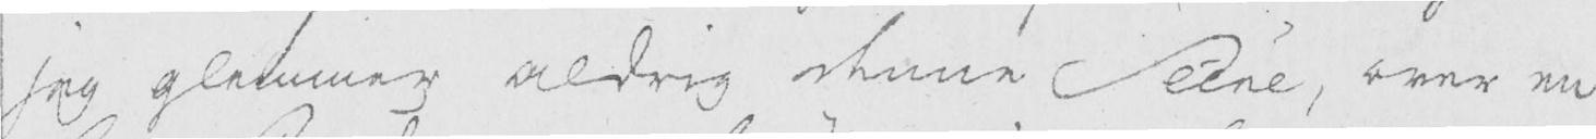jeg glemmer aldrig denne Scene, over en
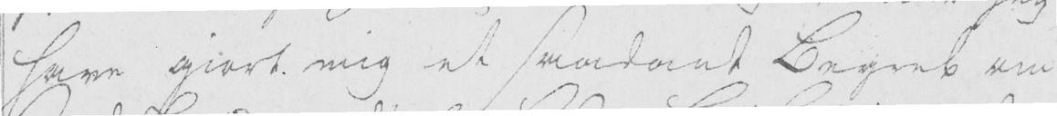have giort mig et saadant Begreb om
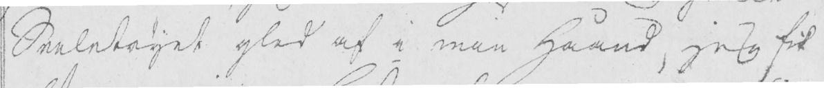Seeletøyet gled af i min Haand, jeg fik
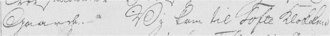Gaarde. Vi kom til Tofte Klokken
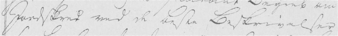Jordskred ved de beste Beskrivelser
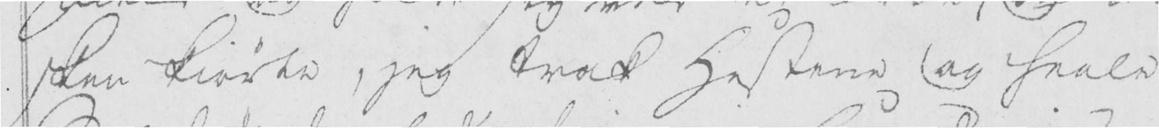sken kiørte, jeg trak Hestene og heele
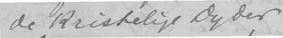de kristelige Dyder.
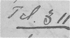Til § 11
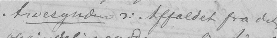Arvesynden ): Affaldet fra det
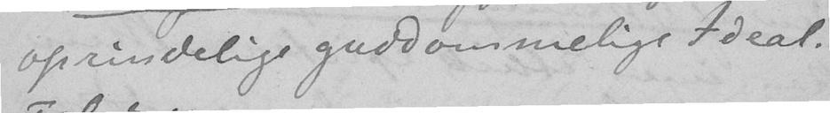oprindelige guddommelige Ideal.
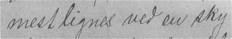mest lignes ved en sky
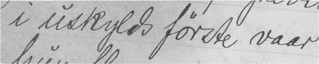i uskylds første vaar
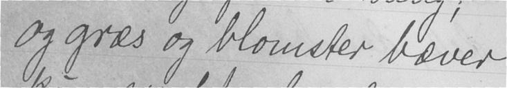og græs og blomster bæver
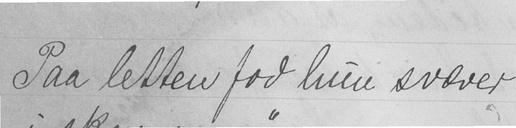Paa letten fod hun svæver
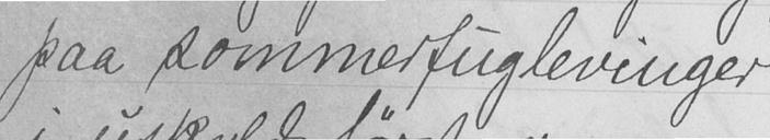paa sommerfuglevinger
